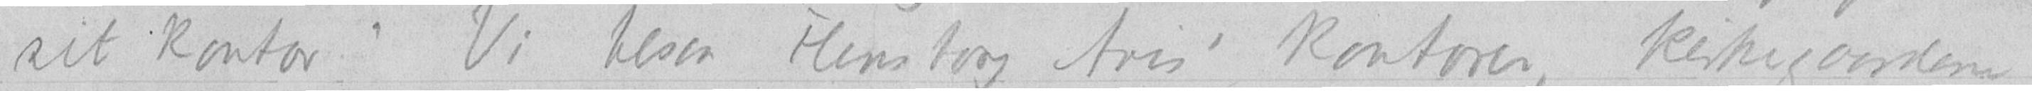sit kontor." Vi besaa Flensborg Aris’ kontorer, kirkegaardene
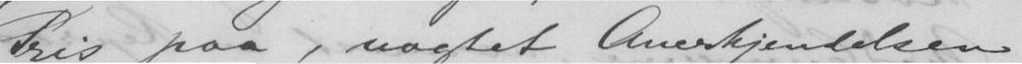Pris paa, uagtet Anerkjendelsen
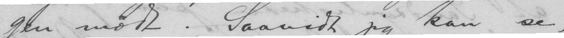gen mødt. Saavidt jeg kan se,
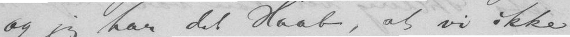og jeg har det Haab, at vi ikke
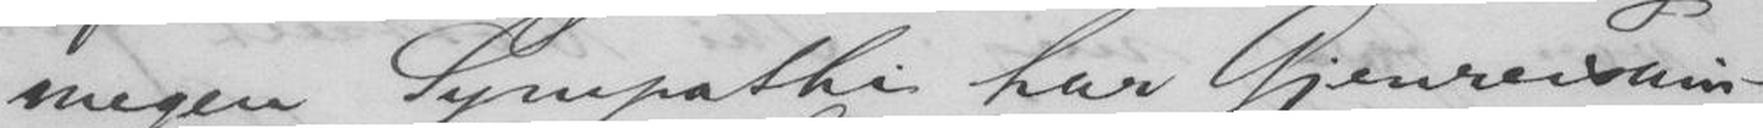megen Sympathi har Gjenreisnin-
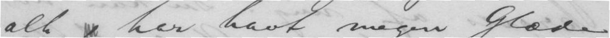alt har havt megen Glæde
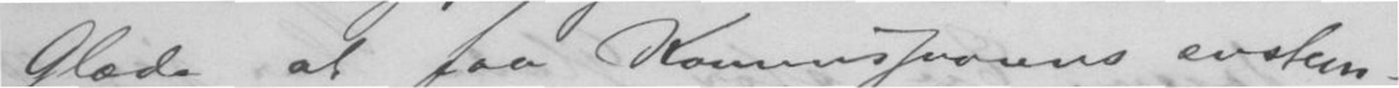Glæde at faa Kommissionens enstem-
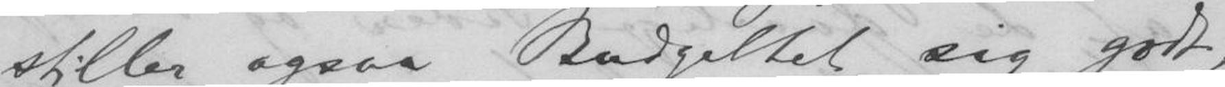stiller ogsaa Budgettet sig godt,
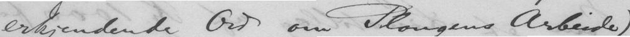erkjendende Ord om Plougens Arbeide)
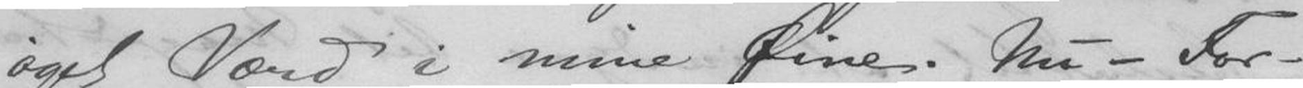øget Værd i mine Øine. Nu - For-
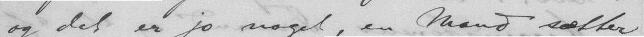og det er jo noget, en Mand sætter
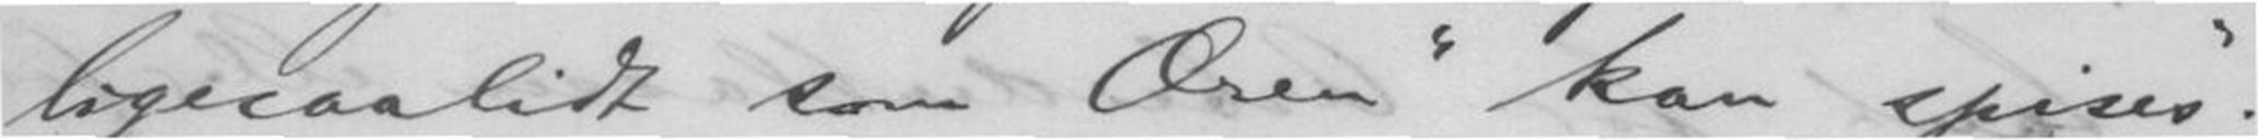ligesaalidt som Æren "kan spises".
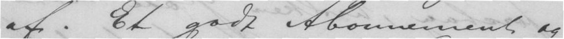af. Et godt Abonnement og
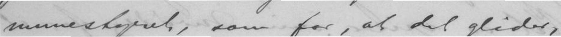munestyret, som for, at det glider, -
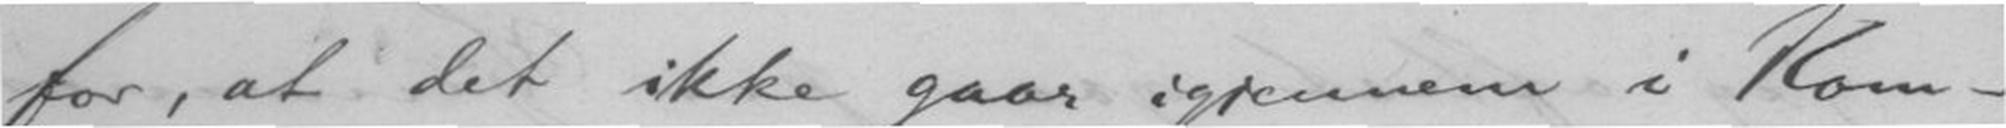for, at det ikke gaar igjennem i Kom-
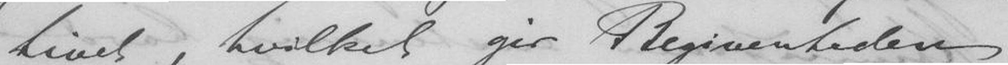tivet, hvilket gir Begivenheden
